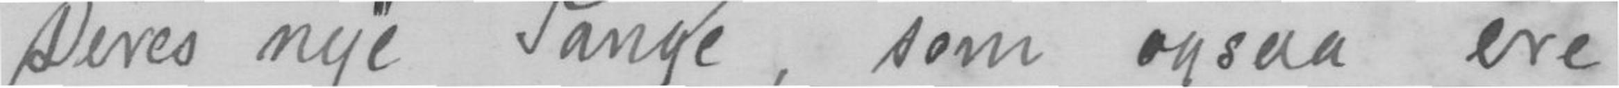Deres nye Sange, som ogsaa ere
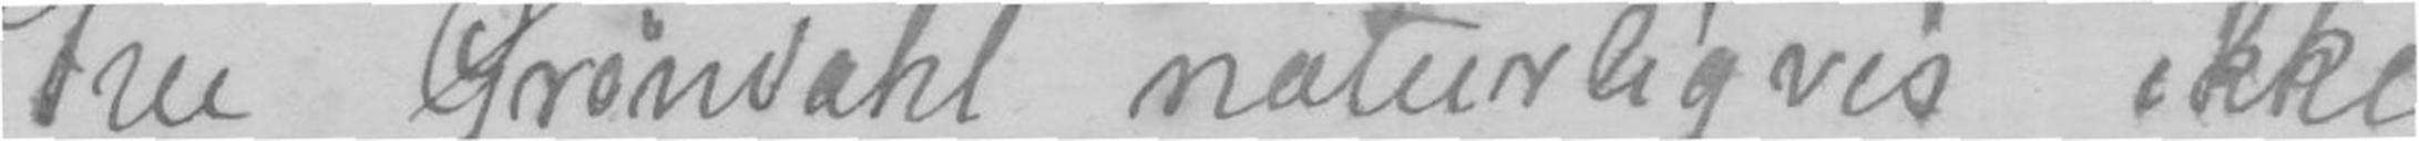Fru Grøndahl naturligvis ikke
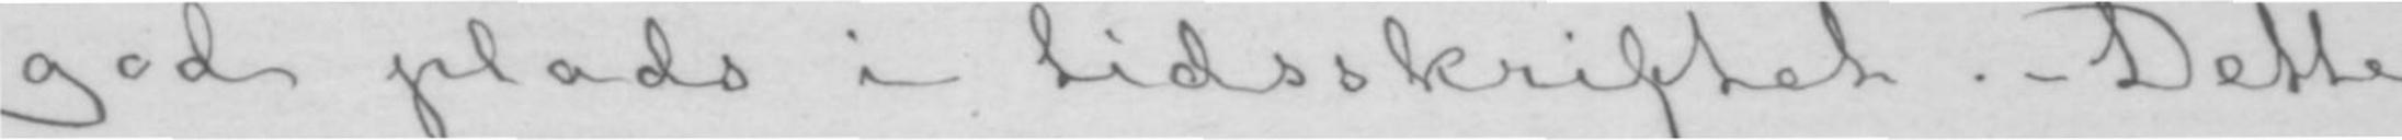god plads i tidsskriftet.- Dette
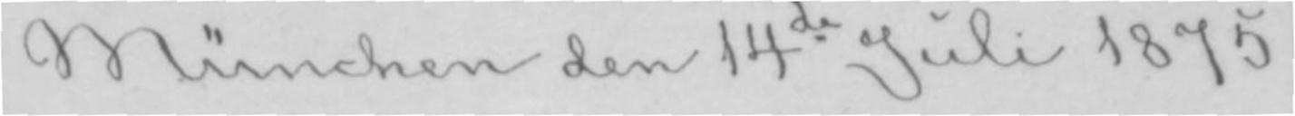München den 14de Juli 1875.
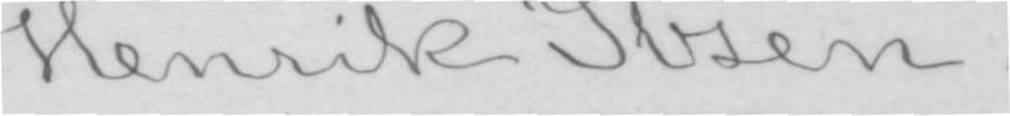Henrik Ibsen.
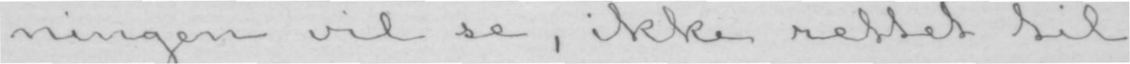ningen vil se, ikke rettet til
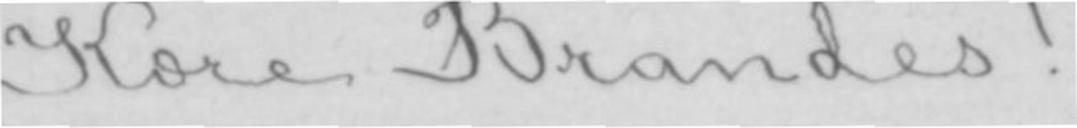Kære Brandes!
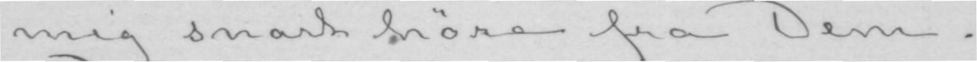mig snart høre fra Dem.
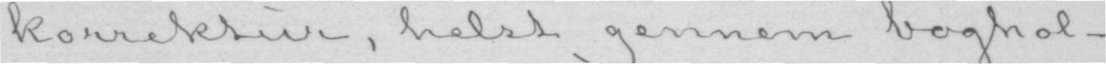korrektur, helst gennem boghol-
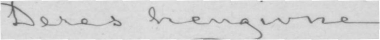Deres hengivne
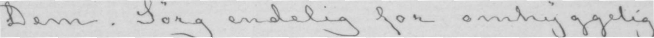Dem. Sørg endelig for omhyggelig
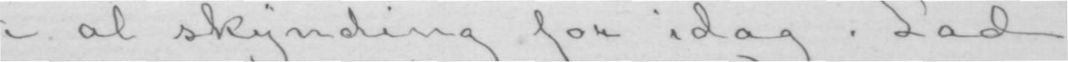i al skynding for idag. Lad
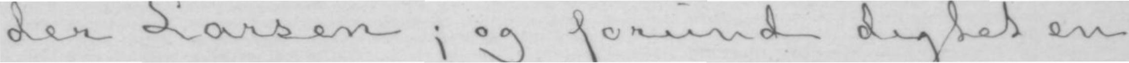der Larsen; og forund digtet en
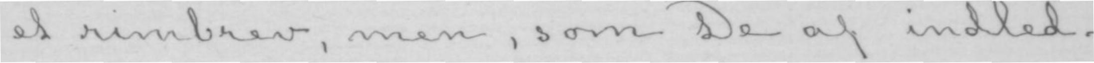et rimbrev, men, som De af indled-
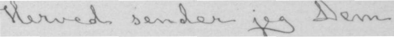Herved sender jeg Dem
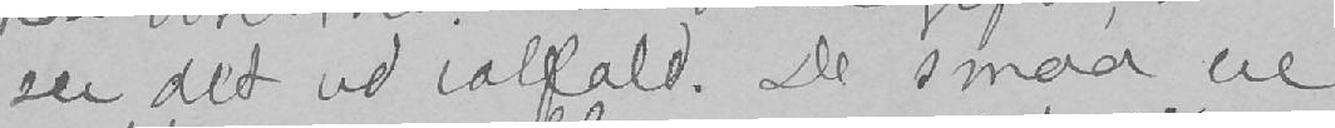ser det ud ialfald. De smaa ere
